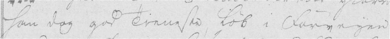han dog god Tieneste, løb i Forveyen
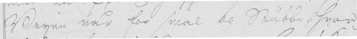Veyen var for smal og Stubbe, hvor
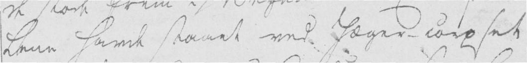lene havde staaet ved Jæger-corpset
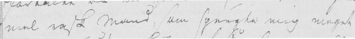mel rask Mand, som spurgte mig meget
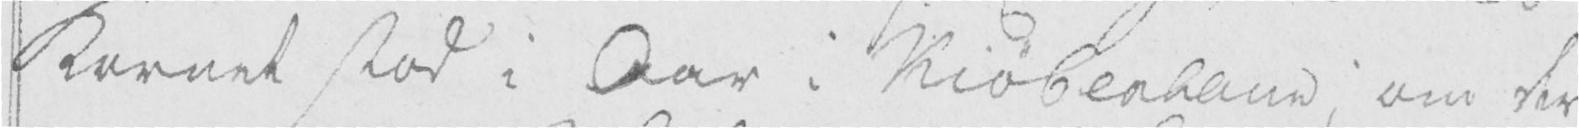Kornet stod i Aar i Kiøbenhavn, om der
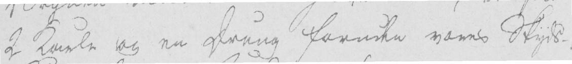2 Karle og en Dreng foruden vores Skyds-
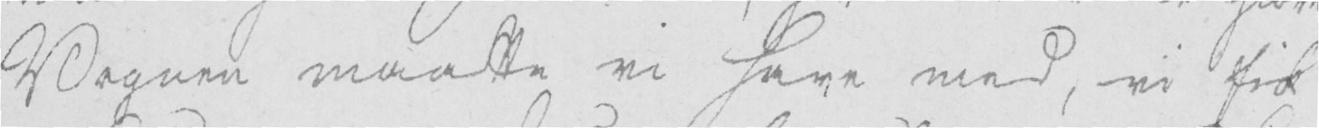Vognen maatte vi have med, vi fik
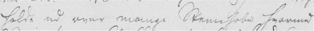holdt ud over mange Steenhobe hvormed
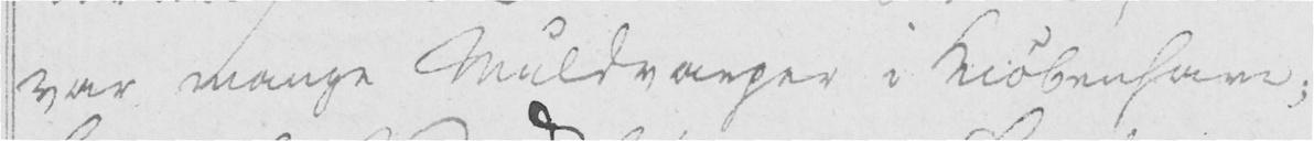var mange muldvarper i Kiøbenhavn;
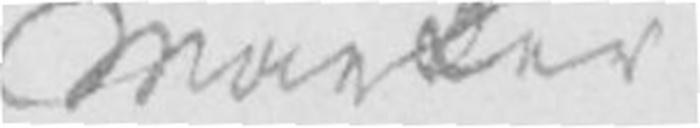Marker
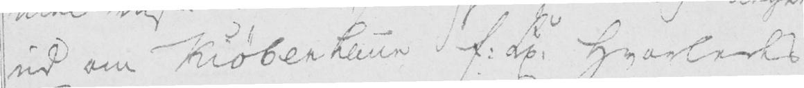ud om Kiøbenhavn f. Ex. Hvorledes
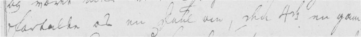fortalte os en Deel om, den 4de en gam-
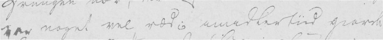var noget vel ræd; imidlertiid giorde
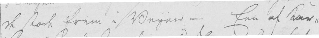de stode frem i Veyen - Een af Kar-
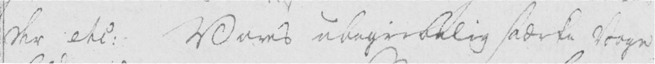der etc. Vores ubegribelig stærke Vogn
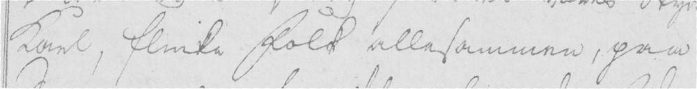karl, flinke Folk allesammen, paa
